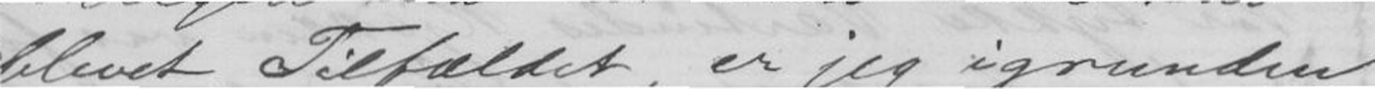blevet Tilfælde, er jeg igrunden
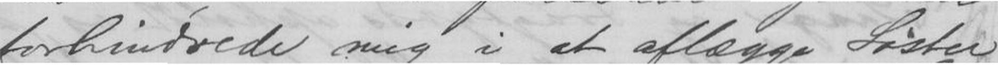forhindrede mig i at aflægge Søster
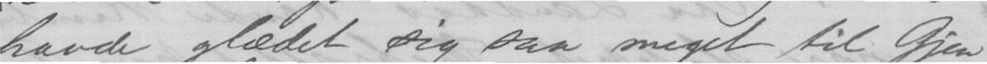havde glædet sig saa meget til Gjen-
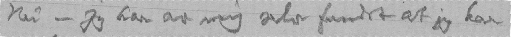Nei - jeg har av mig selv fundet at jeg har
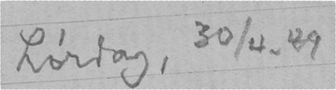Lørdag, 30/4. 49
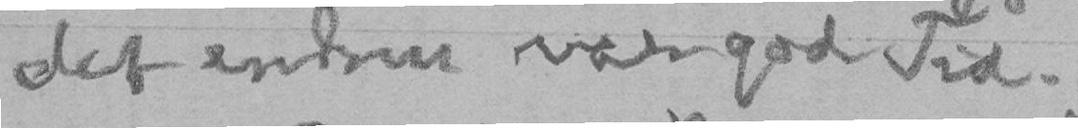det endnu var god Tid.
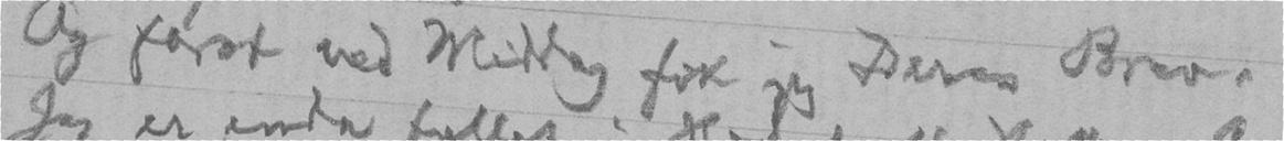Og først ved Middag fik jeg Deres Brev.
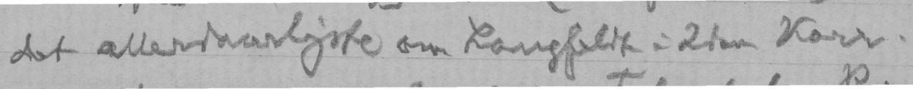det allerdaarligste om Langfeldt i 2den Korr.
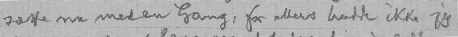sætte nu med en Gang, for ellers hadde ikke jeg
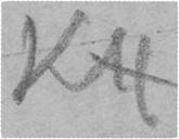KH
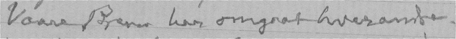Vaare Brever har omgaat hverandre.
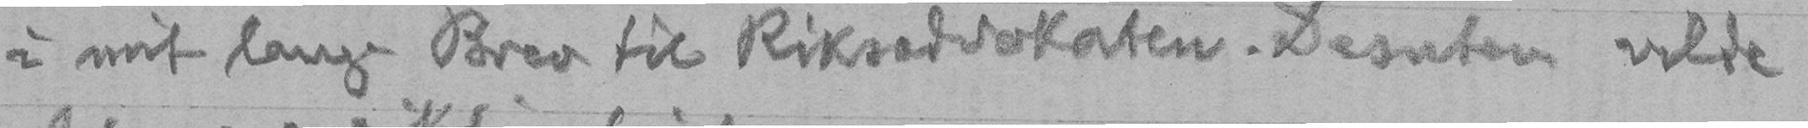i mit lange Brev til Riksadvokaten. Desuten vilde
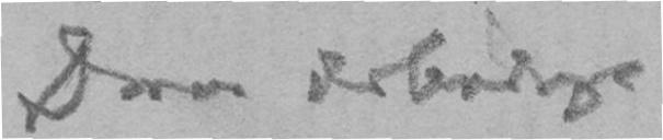Deres ærbødige
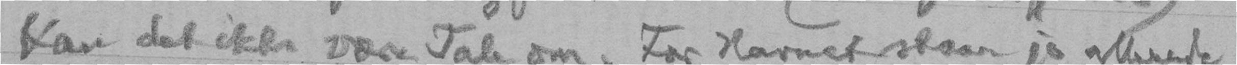kan det ikke være Tale om. For Navnet staar jo allerede
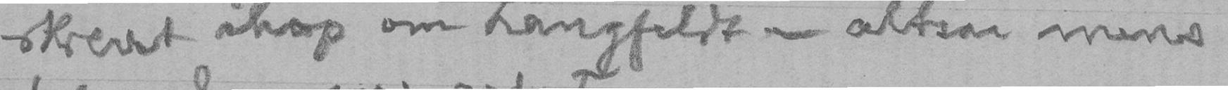skrevet ihop om Langfeldt - altsaa mens
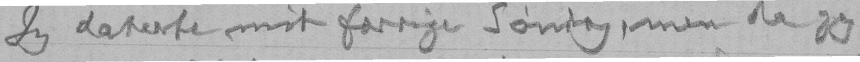Jeg daterte mit forrige Søndag, men da jeg
